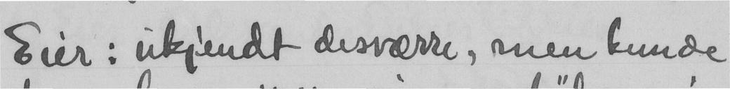Eier: ukjendt desværre, men kunde
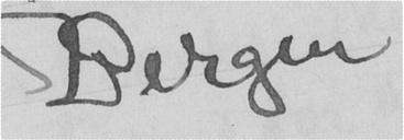Bergen
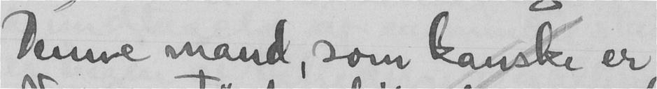Denne mand, som kanske er
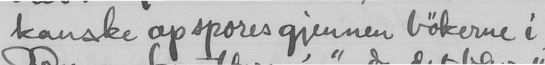kanske opspores gjennen bøkerne i
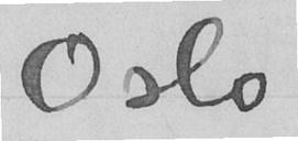Oslo
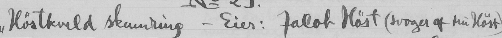"Høstkveld skumring" - Eier: Jacob Høst (svoger af fru Høst)
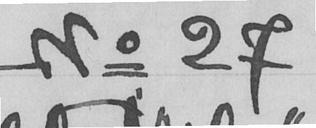No 27
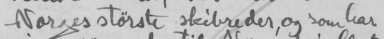Norges største skibreder, og som har
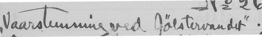"Vaarstemming ved Jølstervandet".
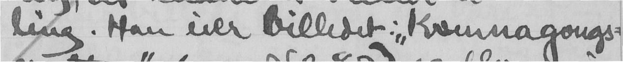ling. Han eier billedet: "Kvennagongs-
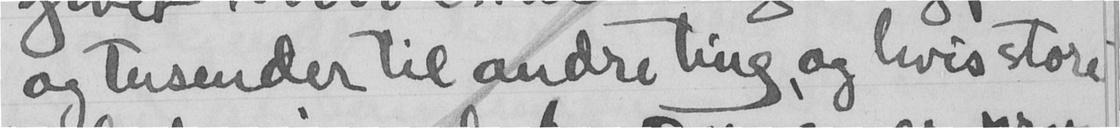og tusender til andre ting, og hvis store
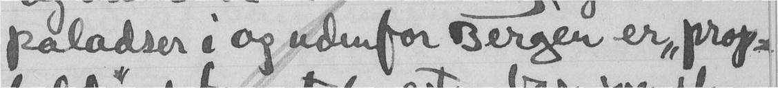paladser i og udenfor Bergen er "prop-
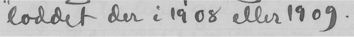loddet der i 1908 eller 1909.
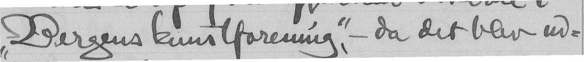"Bergens kunstforening, - da det blev ud-
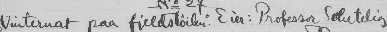"Vinternat paa fjeldstøilen" Eier: Professor Schetelig
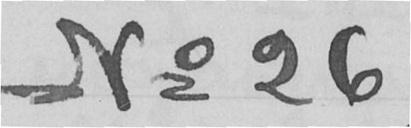No 26
